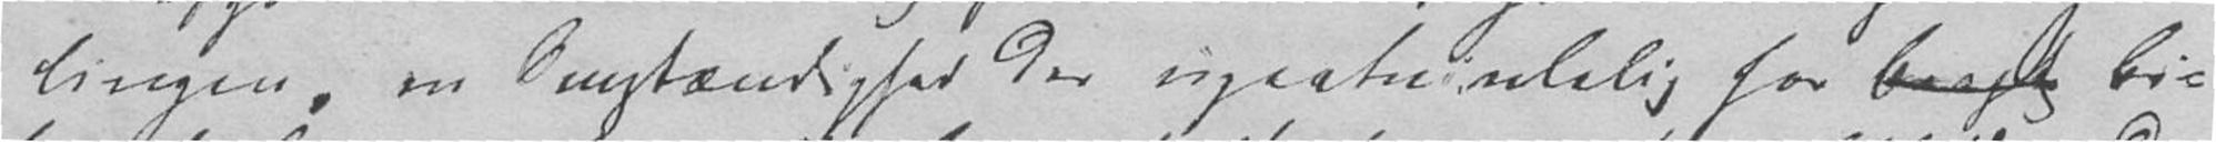lingen, en Omstændighed der upaatvivlelig har bi-
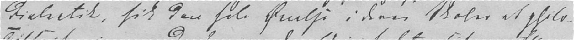Dialectik, fik den hele Øvelse i deres Skoler et philo-
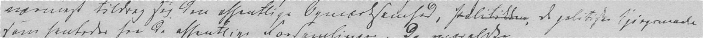nærmest tildrog sig den offentlige Opmærksomhed, de politiske Spørgsmaale
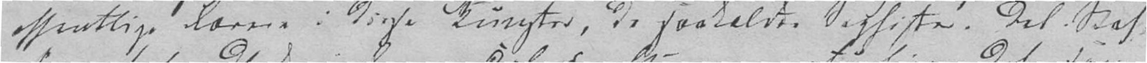offentlige Lærere i disse Kunster, de saakaldte Sophister. Det Stof
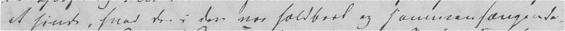at finde, hvad der i den var holdbart og sammenhængende.
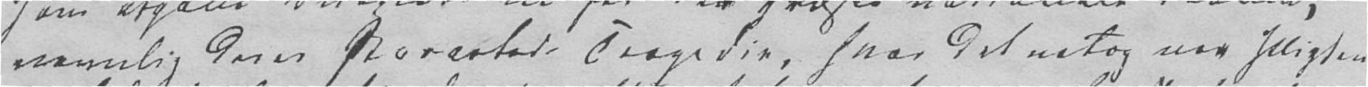nemlig deres storartede Tragedie, hvor det netop var Pligten
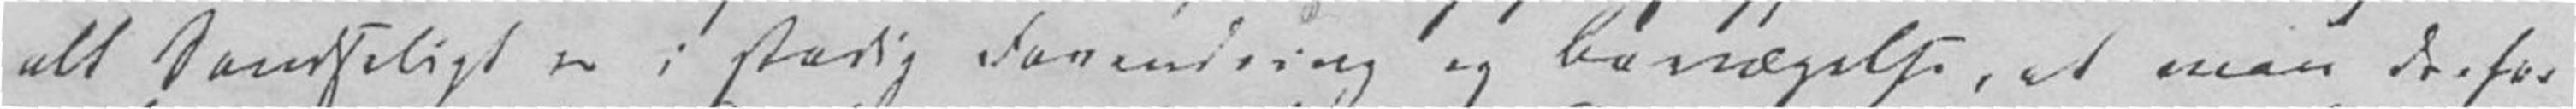alt Sandseligt er i stadig Forandring og Bevægelse, at man derfor
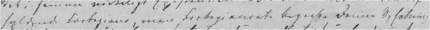fyldende Fortegions, men Fortegionerte Begreb. Denne Opfatning
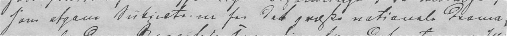som afgav Subjecterne for det græske nationale Drama,
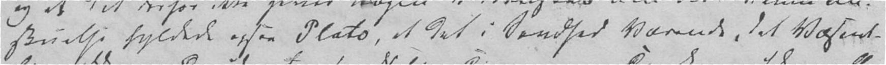skuelse hyldede ogsaa Plato, at det i Sandhed Værende, det Væsent-
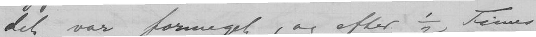det var formeget, og efter ½ Times
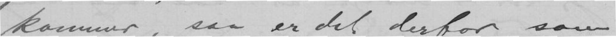kommer, saa er det derfor, som
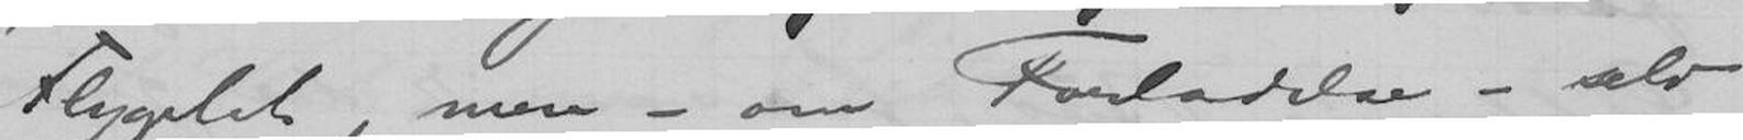Flygelet, men - om Forladelse - selv
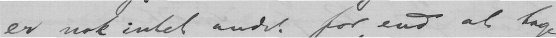er nok intet andet for, end at tage
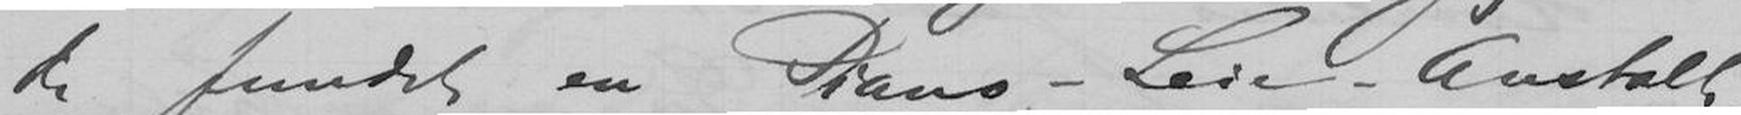de fundet en Piano-Leie-Anstalt,
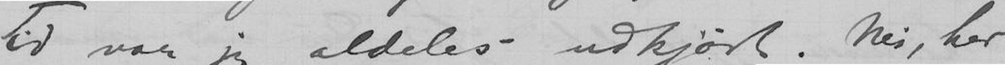Tid var jeg aldeles udkjørt. Nei, her
